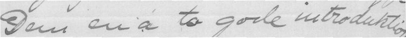Dem en á to gode introduktioner
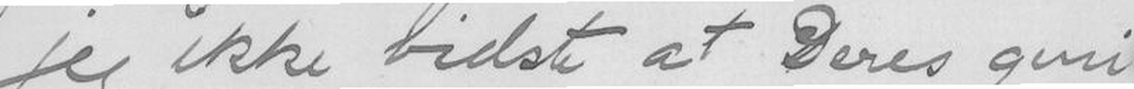jeg ikke vidste at Deres geni
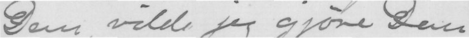Dem, vilde jeg gjøre Dem
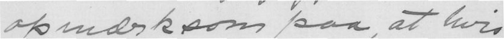opmærksom paa, at hvis
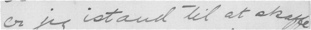er jeg istand til at skaffe
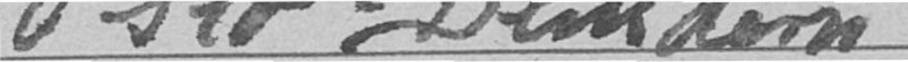Oslo-Blindern
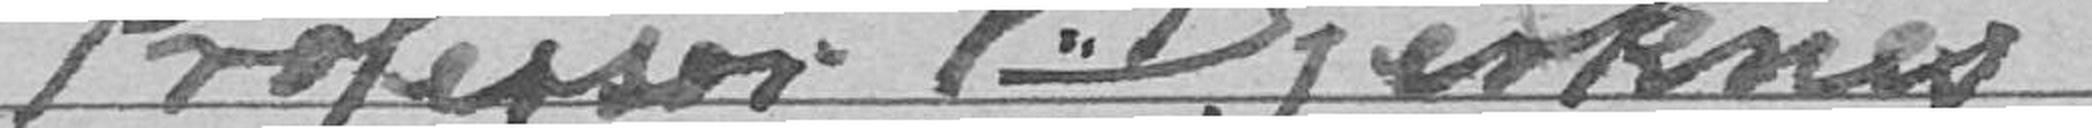Professor V. Bjerknes
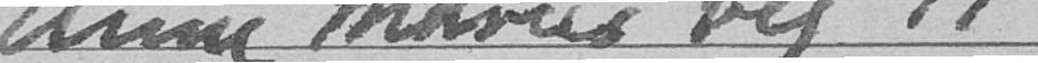Anne Maries vej 14
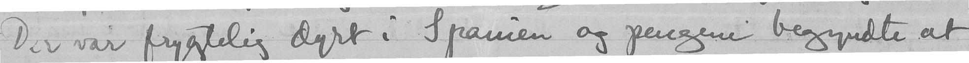Der var frygtelig dyrt i Spanien og pengene begynte at
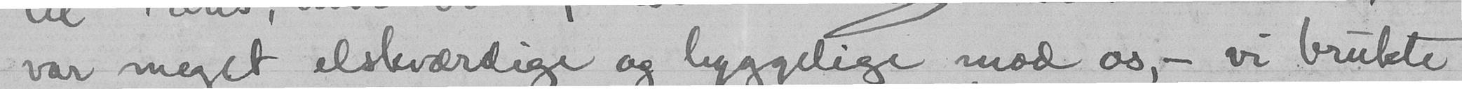var meget elskværdige og lyggelige mod os; - vi brukte
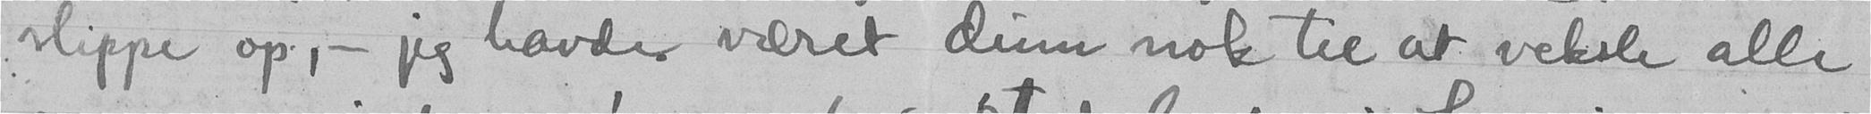slippe op; - jeg havde været dum nok til at veksle alle
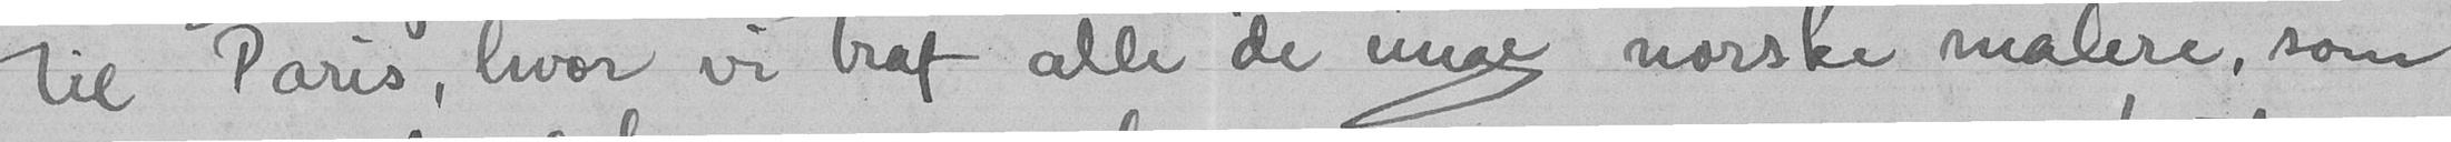til Paris, hvor vi traf alle de unge norske malere, som
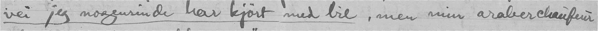vei jeg nogensinde har kjørt med bil, men min araberchaufeur
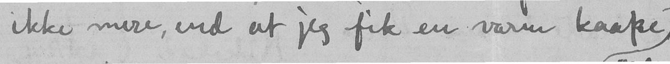ikke mere, end at jeg fik en varm kaape,
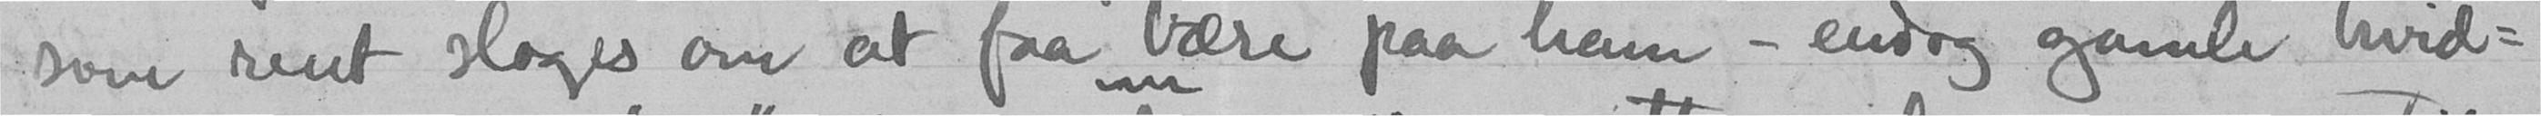som rent sloges om at faa bære paa ham - endog gamle hvid-
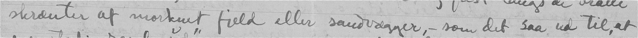skrænter af mørknet fjeld eller sandvægger, - som det saa ud til, at
